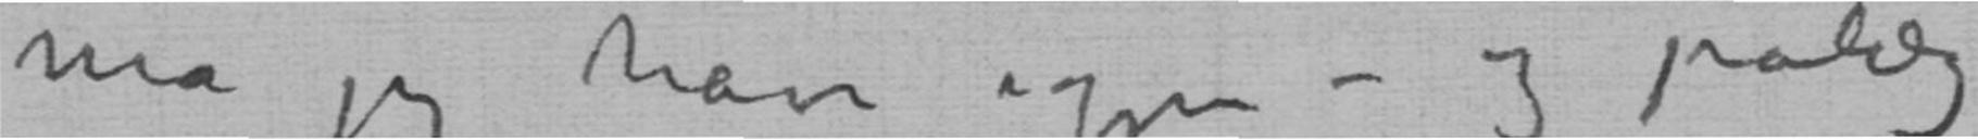må jeg have igjen – og pålæg
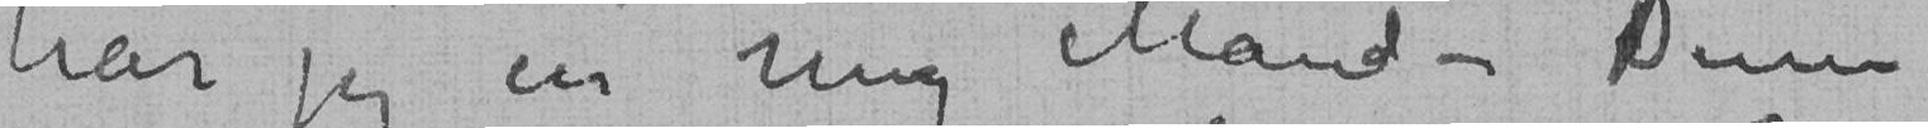har jeg en ung Mand – Denne
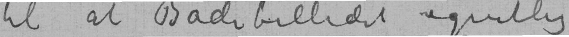til at Badebilledet egentlig
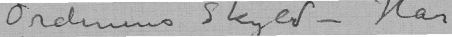Ordenens Skyld – Har
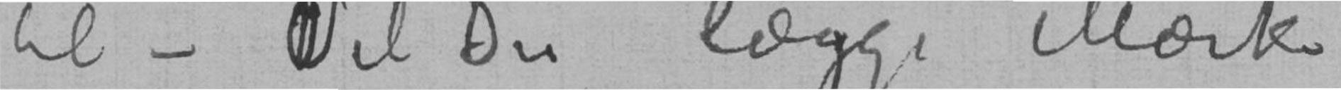til – Vil Du lægge Mærke
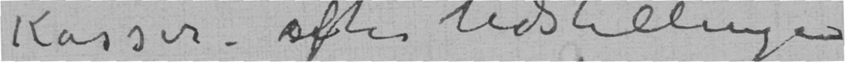Kasser efter Udstillingen
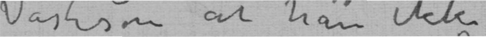Vasteson at han ikke
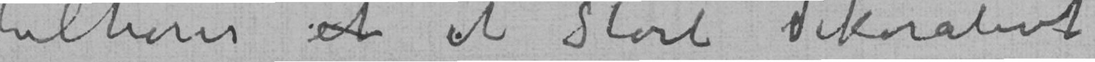tilhører et et stort dekorativt
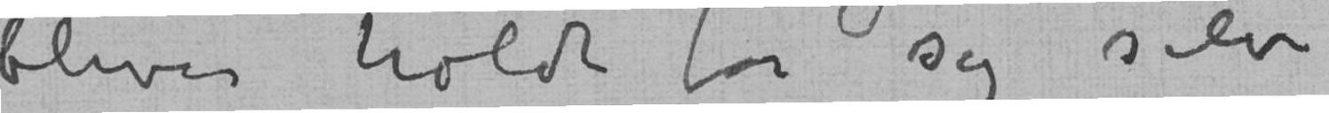bliver holdt for sig selv
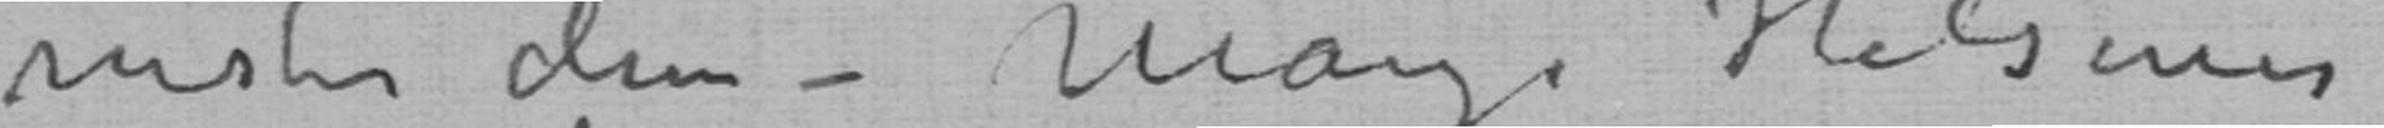mister dem –Mange hilsener
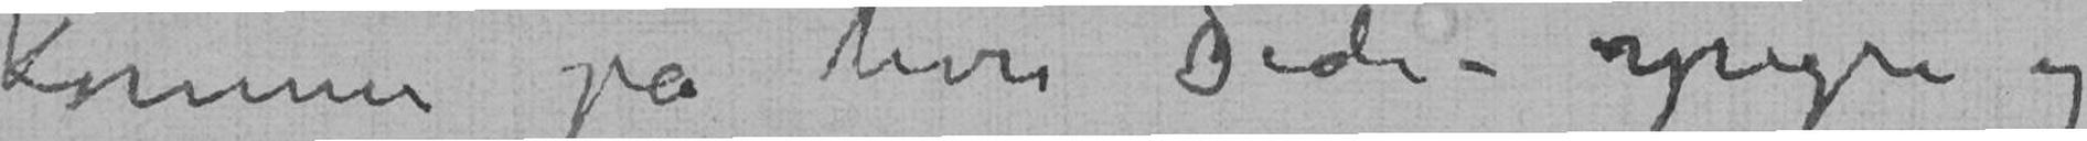kommer på hver Side – yngre og
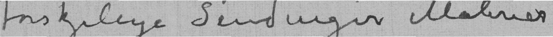forskjelige Sendinger Malerier
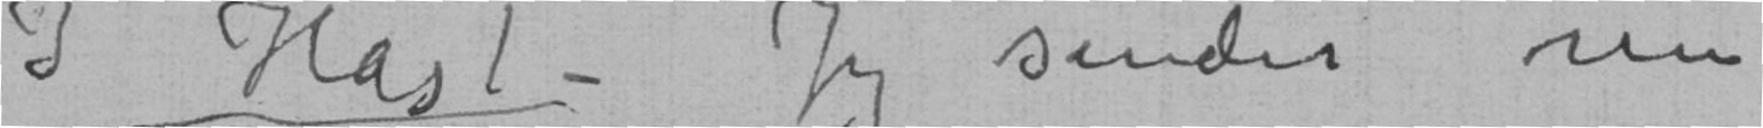I Hast – Jeg sender nu
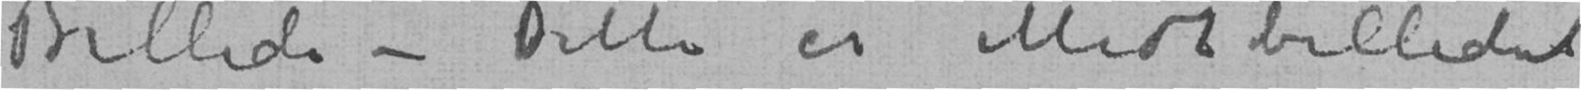Billede – Dette er Midtbilledet
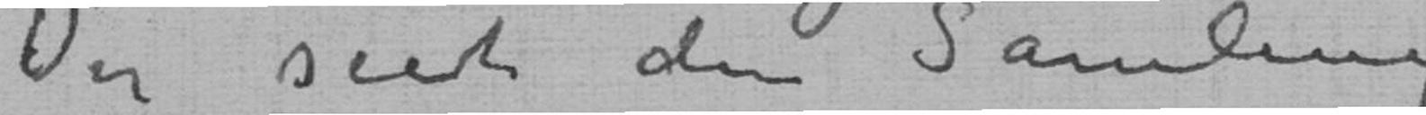Du seet den Samling
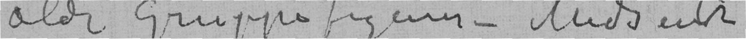ældre Gruppefigurer – Medsendt
